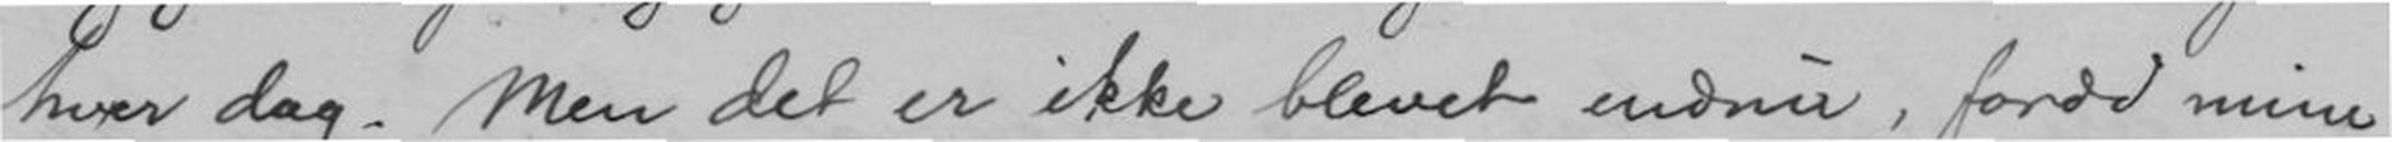hver dag. Men det er ikke blevet endnu, fordi mine
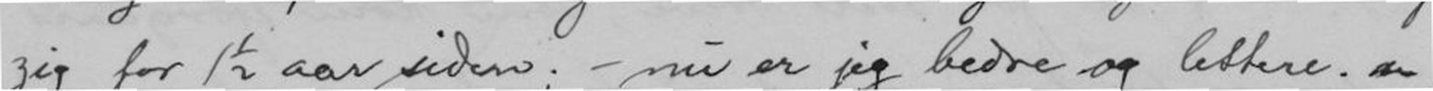zig for 1 1/2 aar siden; - nu er jeg bedre og lettere.
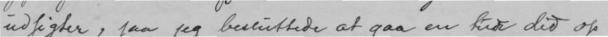udsigter, saa jeg besluttede at gaa en tur did op
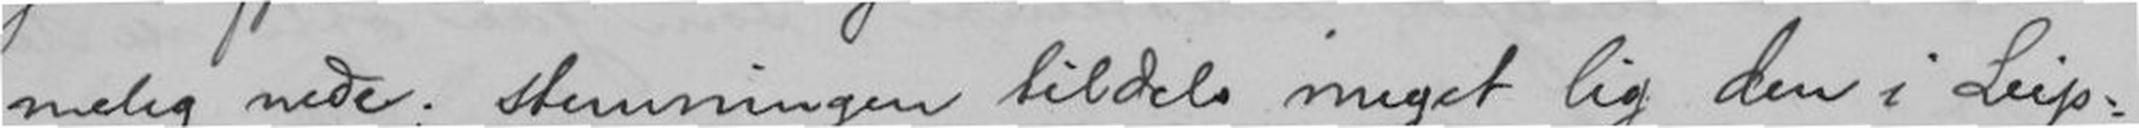melig nede; stemningen tildels meget lig den i Leip-
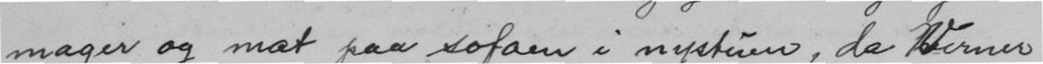mager og mat paa sofaen i nystuen, da Verner
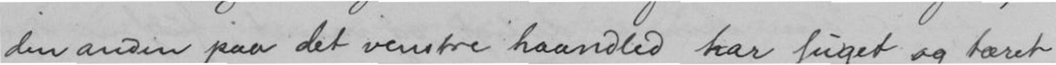den anden paa det venstre haandled har suget og tæret
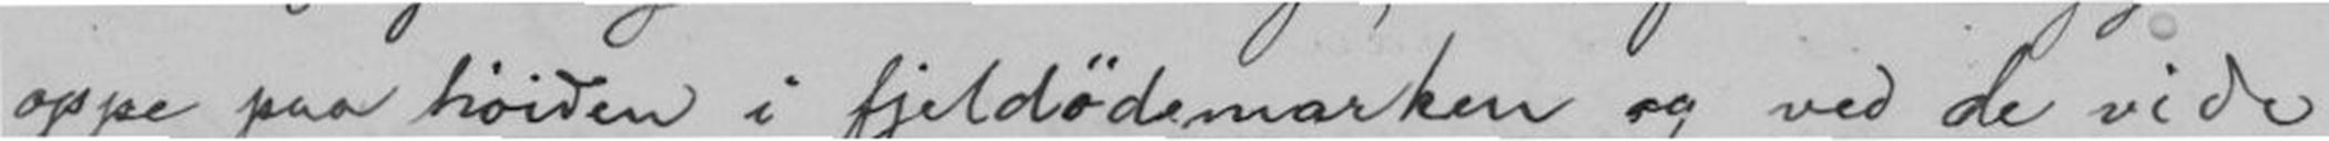oppe paa høiden i fjeldødemarken og ved de vide
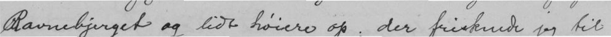Ravnebjerget og lidt høiere op; der frisknede jeg til
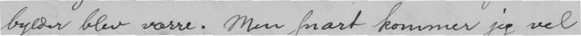bylderblev værre. Men snart kommer jeg vel
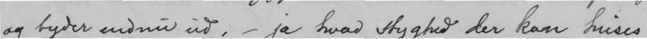og tyder endnu ud, - ja hvad styghed der kan huses
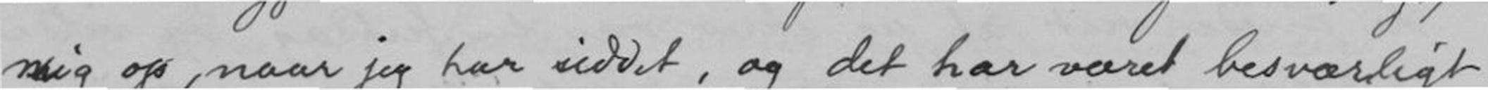mig op naar jeg har siddet, og det har været besværligt
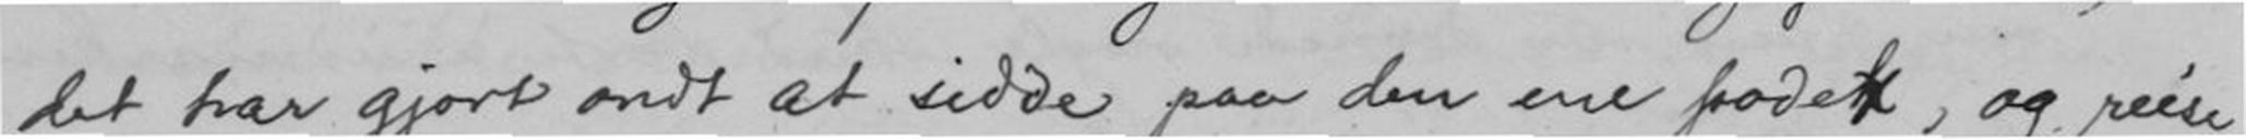det har gjort ondt at sidde paa den ene pode, og reise
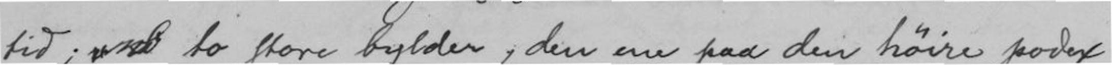tid; to store bylder, den ene paa den høire pode-
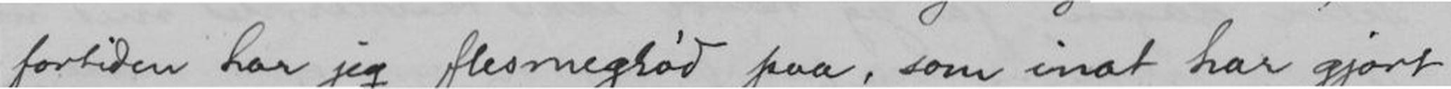fortiden har jeg flesmegrød paa, som inat har gjort
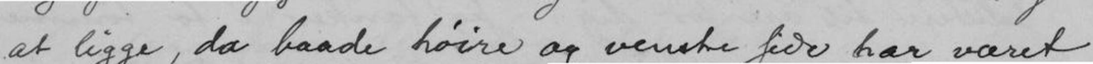at ligge, da baade høire og venstre side har været
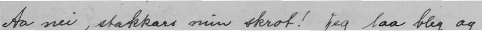Aa nei, stakkars min skrot! Jeg laa bleg og
